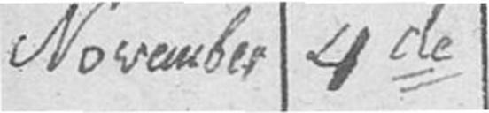November 4de
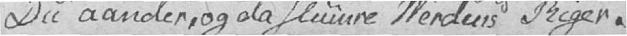Du aander, og da slumre Verdens Riger.
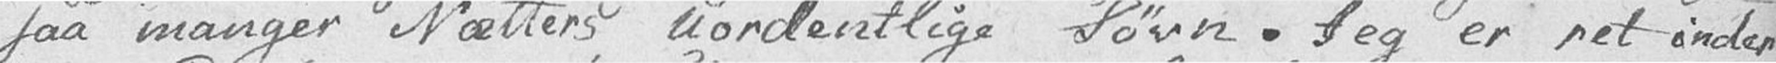saa manger Nætters uordentlige Søvn. Jeg er ret inder
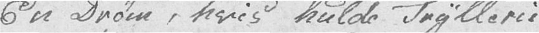En Drøm, hvis hulde Tryllerie
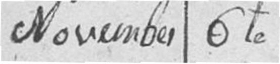November 6te
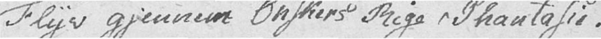Flyv gjennem Ønskers Rige Phantasie!
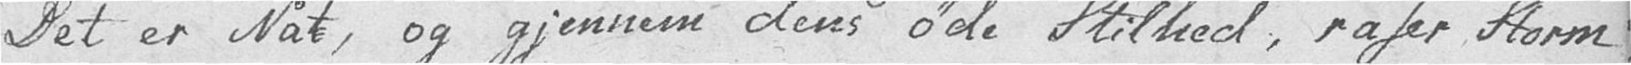Det er Nat, og gjennem dens øde Stilhed, raser Storm
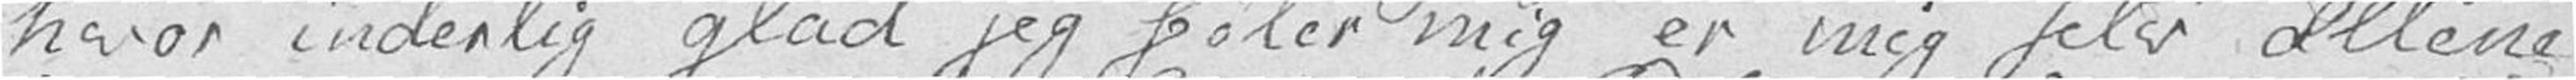hvor inderlig glad jeg føler mig er mig selv allene
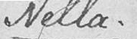Nella
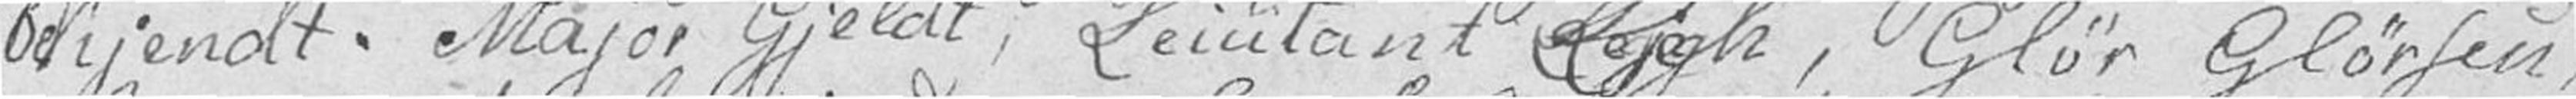bekjendt. Major Gjeldt, Leiutant Leigh, Glør Glørsen,
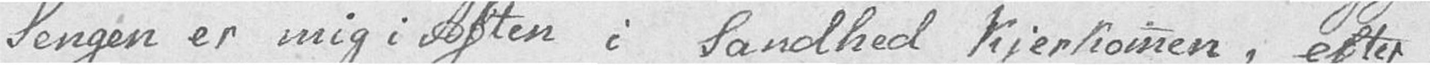Sengen er mig i Aften i Sandhed kjerkommen, efter
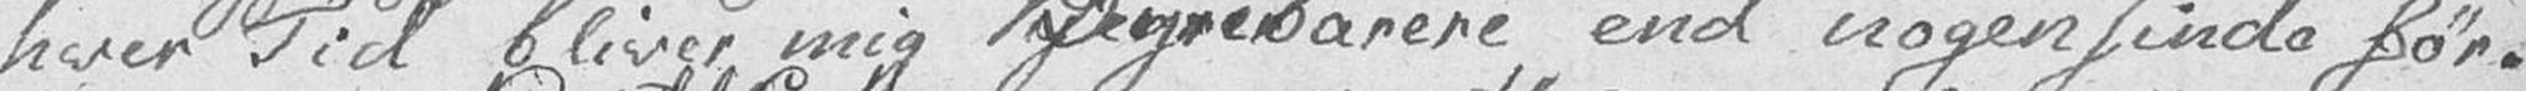hver Tid bliver mig Dyrebarere end nogensinde før.
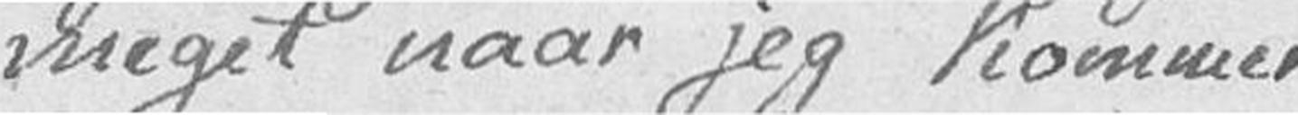meget naar jeg kommer
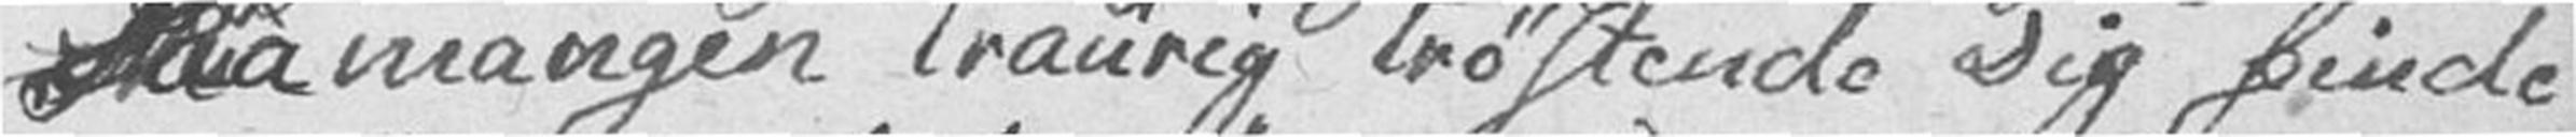Saa mangen traurig trøstende Dig finde
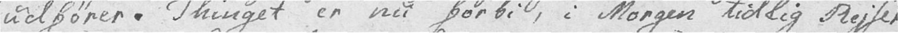udfører. Thinget er nu forbi, i Morgen tidlig Rejser
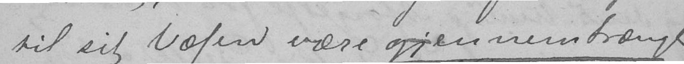til sit Væsen være gjennemtrængt
